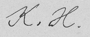K. H.
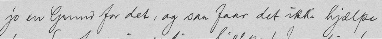jo en Grund for det, og saa faar det ikke hjælpe
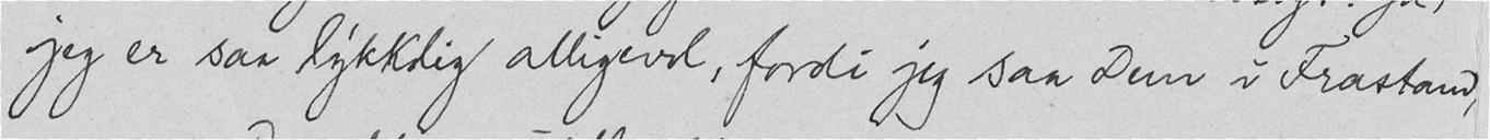jeg er saa lykkelig alligevel, fordi jeg saa Dem i Frastand,
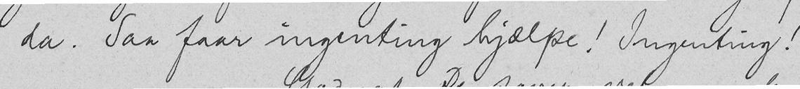da. Saa faar ingenting hjælpe! Ingenting!
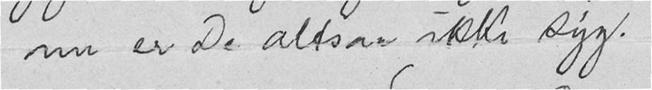nu er De altsaa ikke syg.
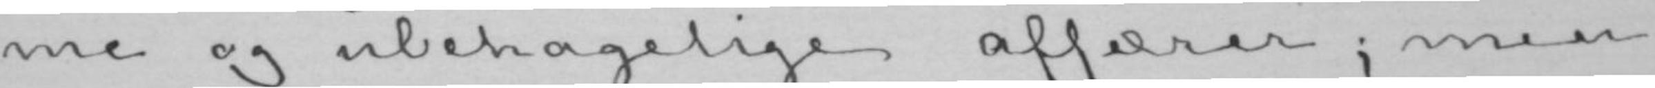me og ubehagelige affærer; men
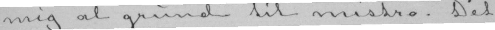mig al grund til mistro. Det
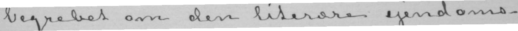begrebet om den literære ejendoms-
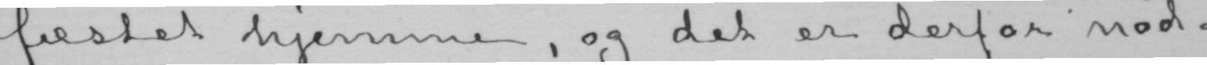fæstet hjemme, og det er derfor nød-
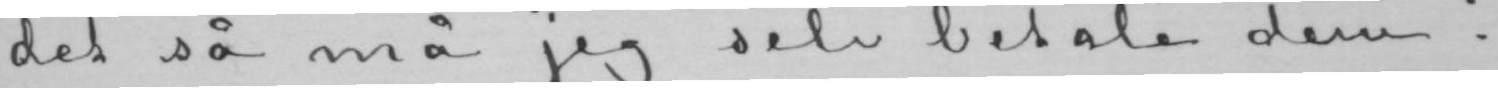det så må jeg selv betale dem.
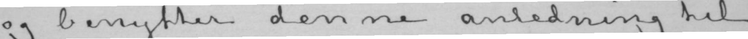og benytter denne anledning til
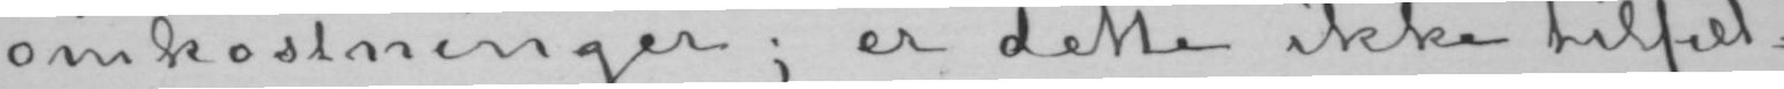omkostninger; er dette ikke tilfæl-
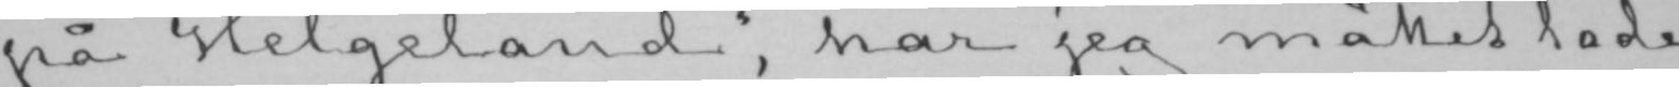på Helgeland», har jeg måttet lade
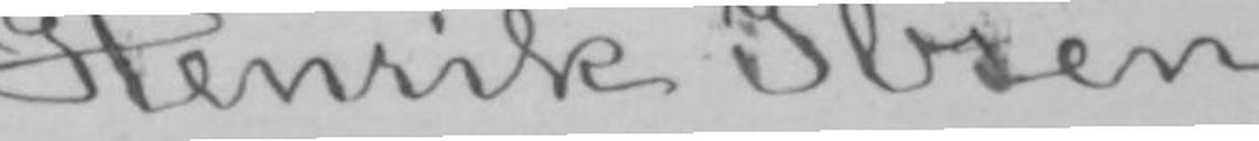Henrik Ibsen.
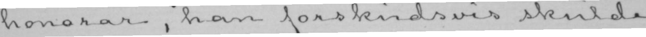honorar, han forskudsvis skulde
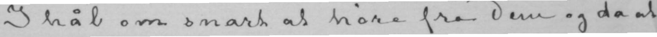I håb om snart at høre fra Dem og da at
